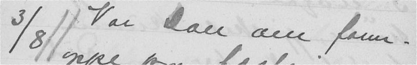3/8 Var Don om form.
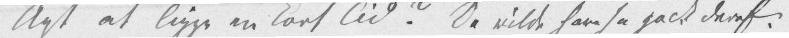Agt at ligge en kort Tid? De vilde have så godt deraf.
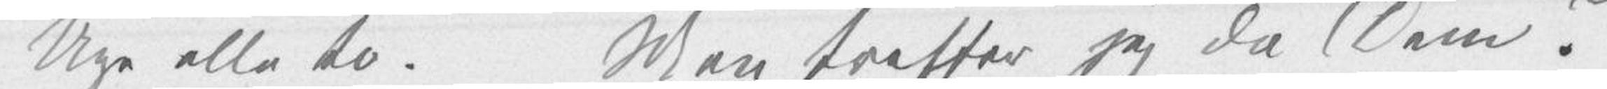Uge eller to. Men treffer jeg da Dem?
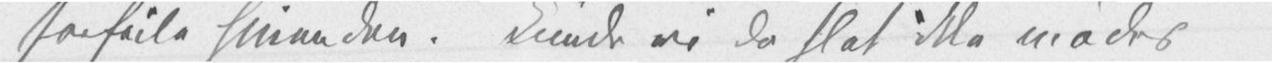forfeile hinanden. Kunde vi da slet ikke mødes
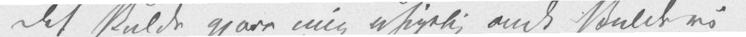Det skulde gjøre mig uhyrlig ondt skulde vi
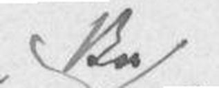ske
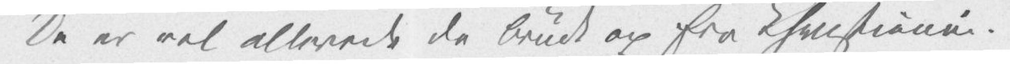De er vel allerede da brudt op fra Christiania.
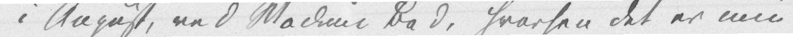i August, ved Modum Bad, hvorhen det er min
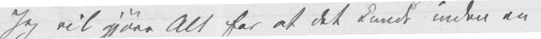Jeg vil gjøre Alt for at det kunde inden en
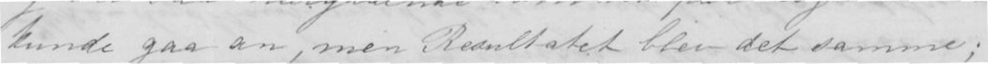kunde gaa an, men Resultatet blev det samme;
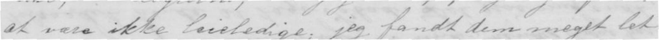at være ikke leieledige; jeg fandt dem meget let
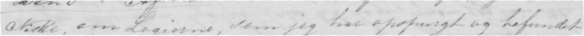Niske, om Logierne, som jeg har opspurgt og befundet
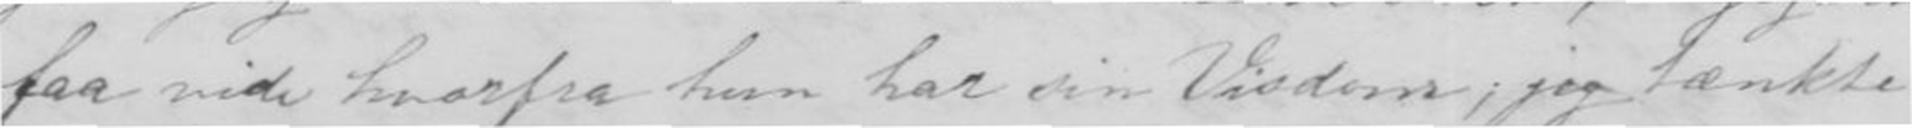faa vide hvordan hun har sin Visdom; jeg tænkte
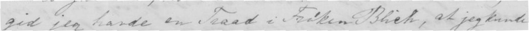gid jeg havde en Traad i Frøken Blich, at jeg kunde
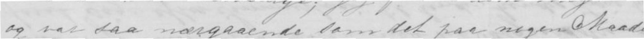og var saa nærgaaende som det paa nogen Maade
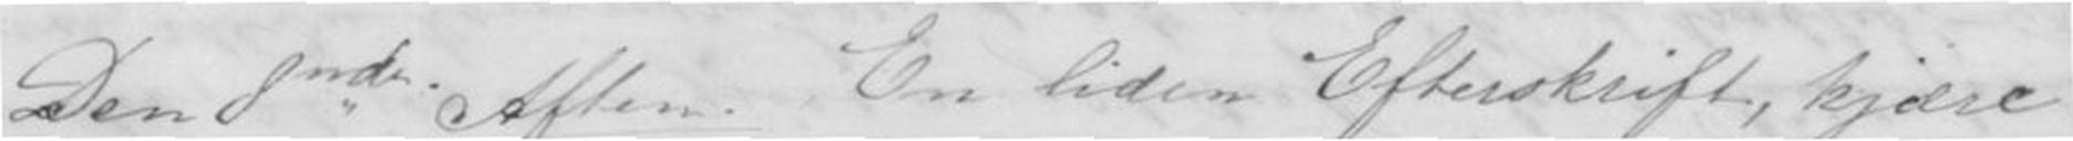Den 8nde Aften. En liden Efterskrift, kjære
